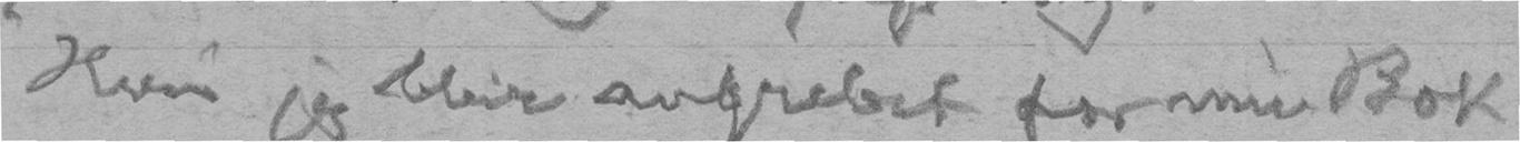Hvis jeg blir angrebet for min Bok
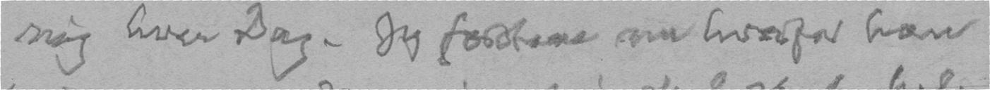mig hver Dag. Jeg forstaar nu hvorfor han
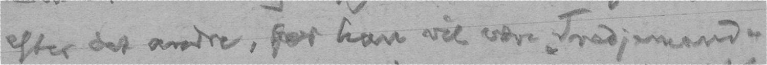efter det andre, for han vil være "Tredjemand"
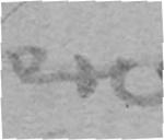en
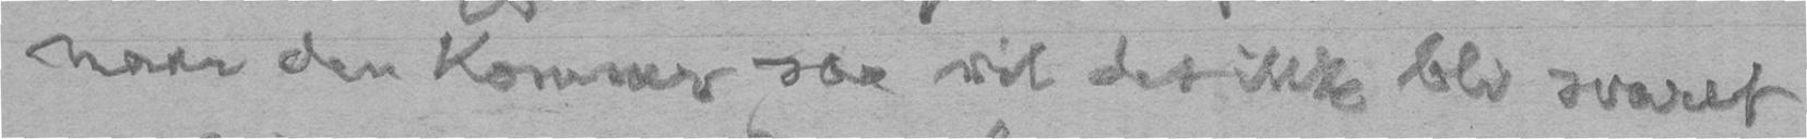naar den kommer saa vil det ikke bli svaret
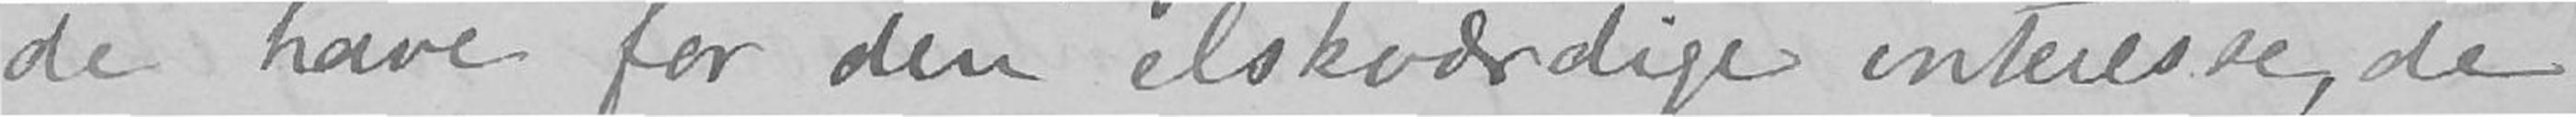de have for den elskværdige interessse, de
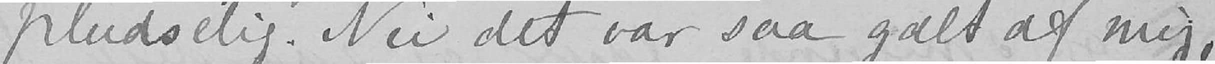pludselig. Nei det var saa galt af mig,
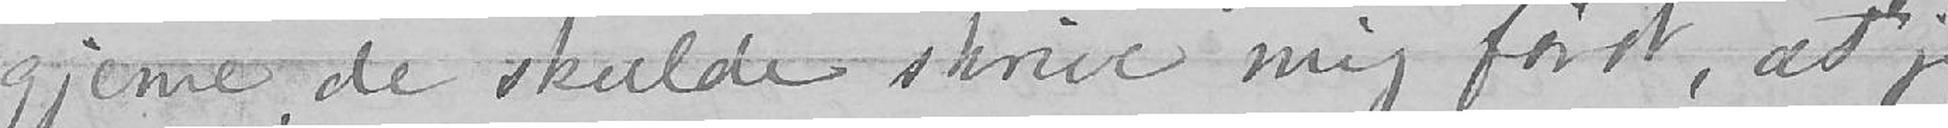gjerne, de skulde skrive mig først, at jeg
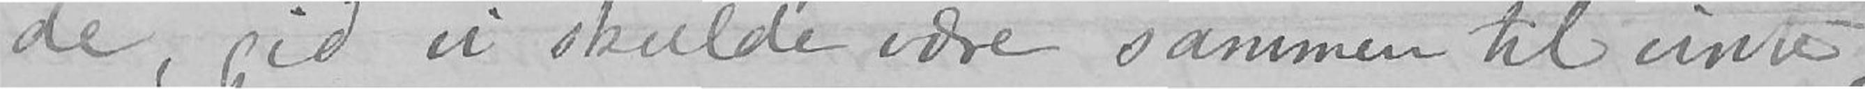de, gid vi skulde være sammen til vinte-
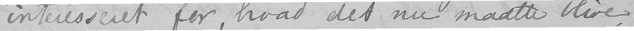interesseret for, hvad det nu maatte blive.
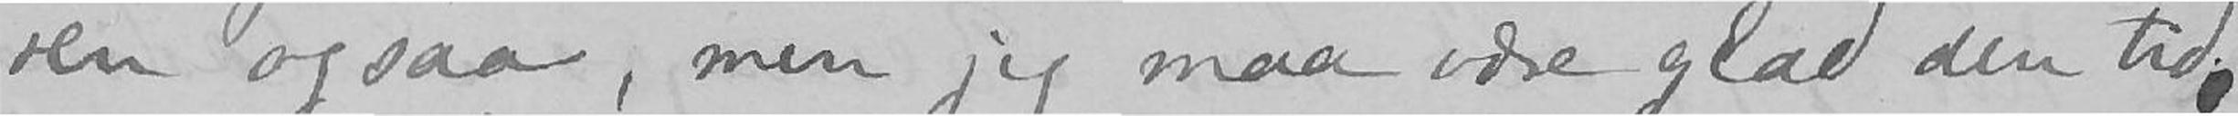ren ogsaa, men jeg maa være glad den tid
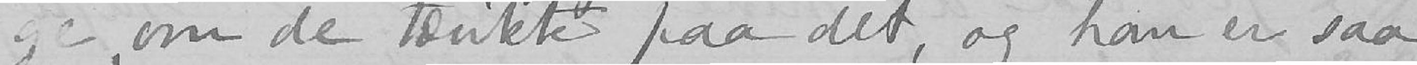ge, om de tænkte paa det, og han er saa
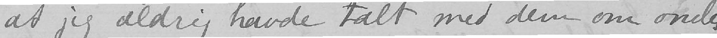at jeg aldrig havde talt med dem om oncles
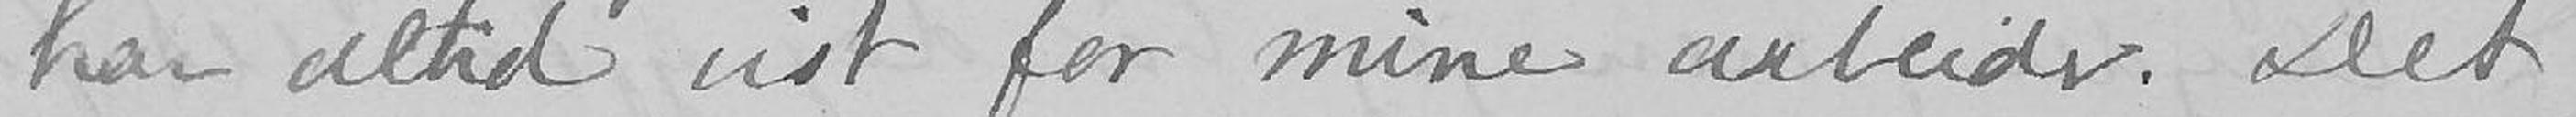har altid vist for mine arbeider. Det
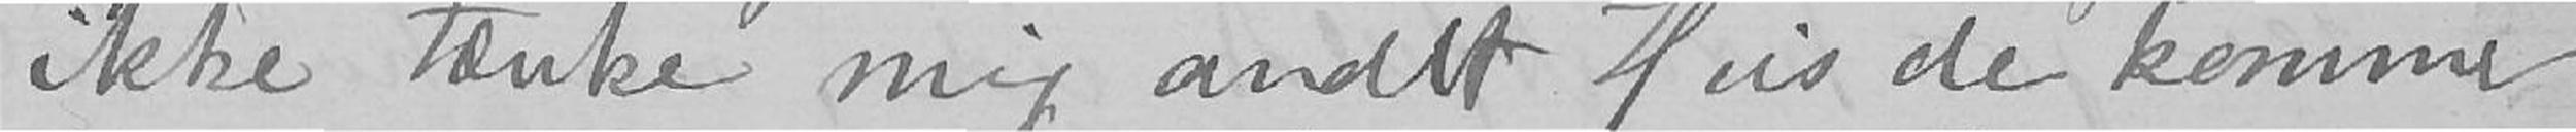ikke tænke mig andet Hvis de kommer
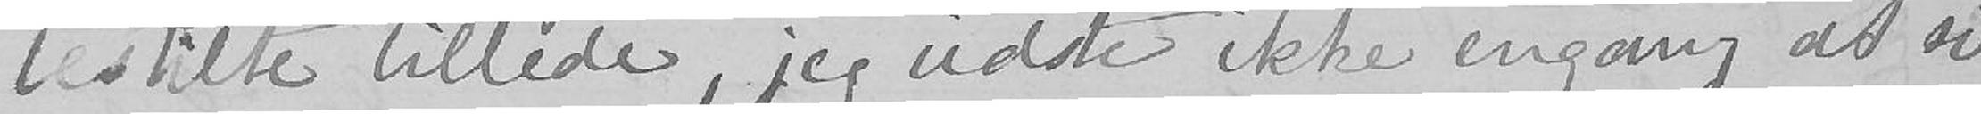bestilte billede, jeg vidste ikke engang at si-
